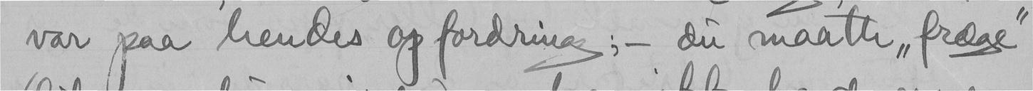var paa hendes opfordring; - du maatte "fræge"
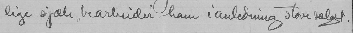lige sjæle "bearbeider" ham i anledning stovesalget.
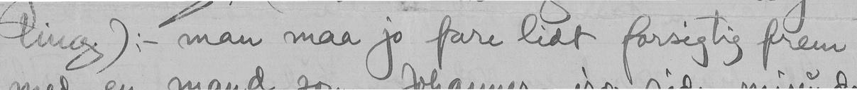ting), - man maa jo fare lidt forsigtig frem
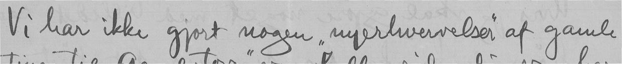Vi har ikke gjort nogen "nyerhvervelser" af gamle
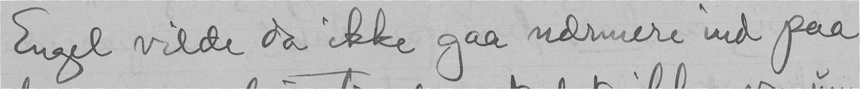Engel vilde da ikke gaa nærmere ind paa
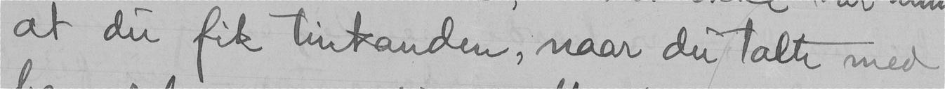at du fik tinkanden, naar du talte med
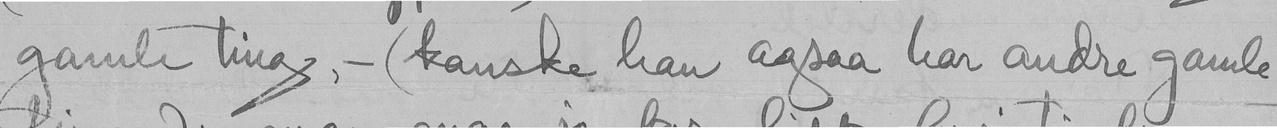gamle ting, - (kanske han ogsaa har andre gamle
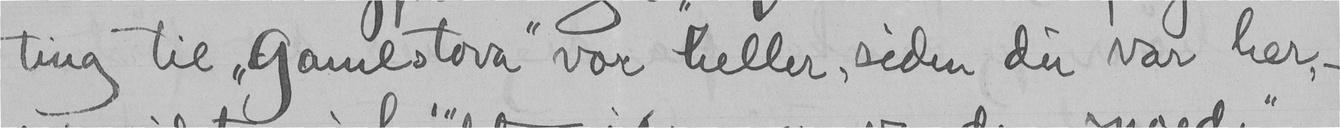ting til "gamestova" vor heller, siden du var her, -
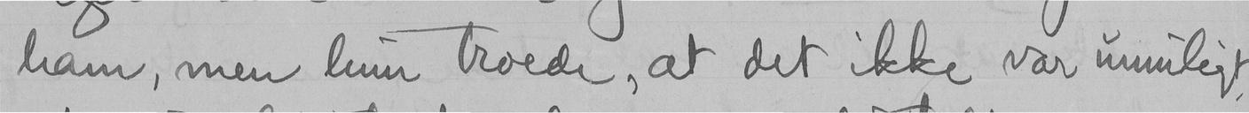ham, men hun troede, at det ikke var umuligt,
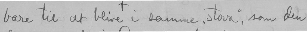bare til at blive i samme "stova", som den
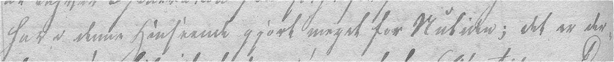har i denne Henseende giort meget for Nutiden; det er der-
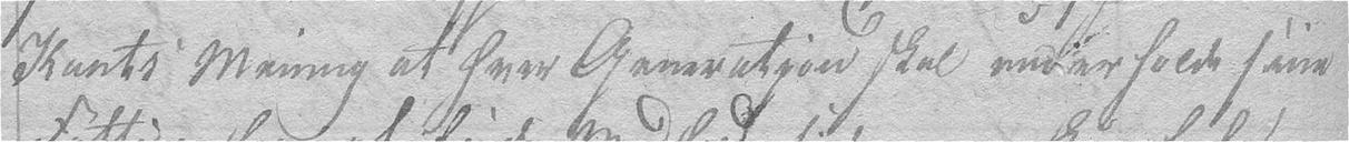Kants Mening at hver Generation skal underholde sine
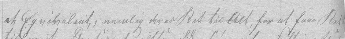et Eqvivalent, nemlig deres Ret til Alt, for at faae Ret
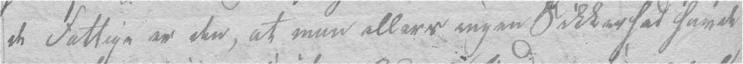de Fattige er den, at man ellers ingen Sikkerhed havde
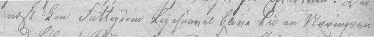næst kan Fattigdom ligesaavel blive til en Næringsvei
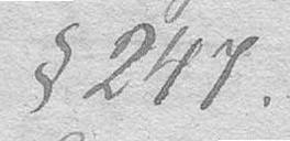§ 247.
